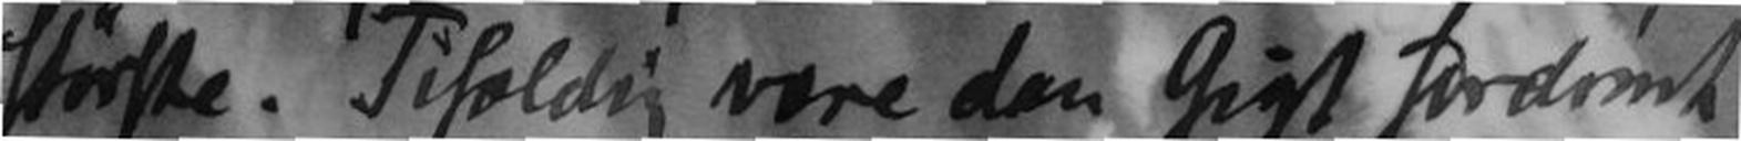største. Tifældig være den Gigt fordømt
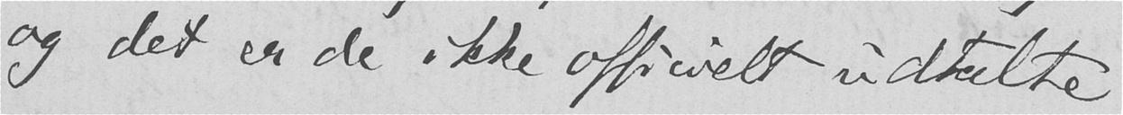og det er de ikke officielt udtalte
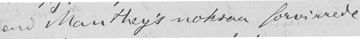end Manthey's noksaa forvirrede
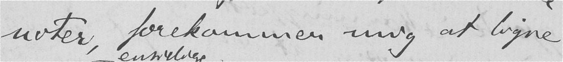noter, forekommer mig at ligne
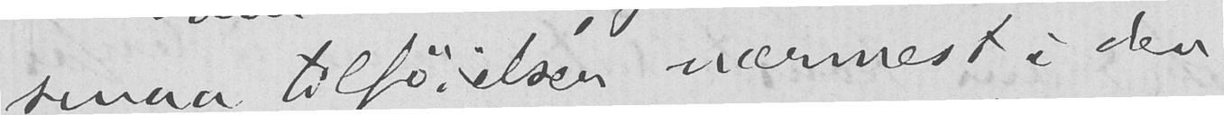smaa tilføielser nærmest i den
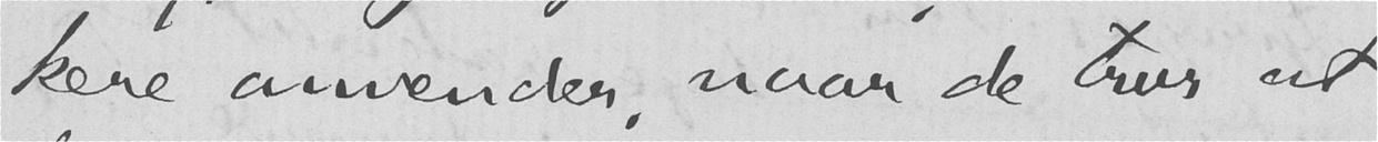kere anvender, naar de tror at
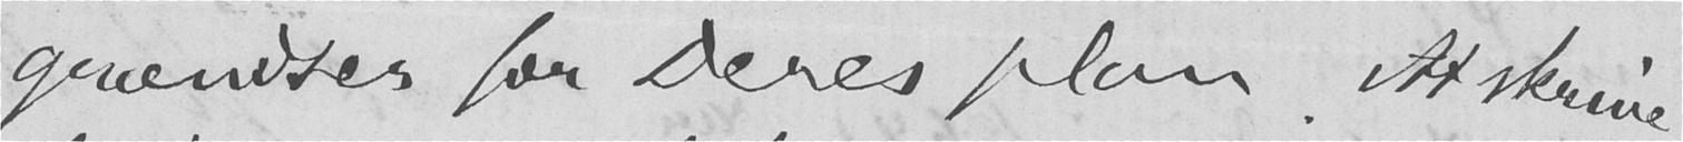grændser for Deres plan. At skrive
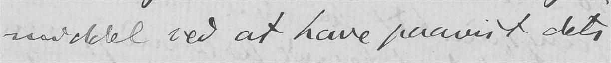middel ved at have paavist dets
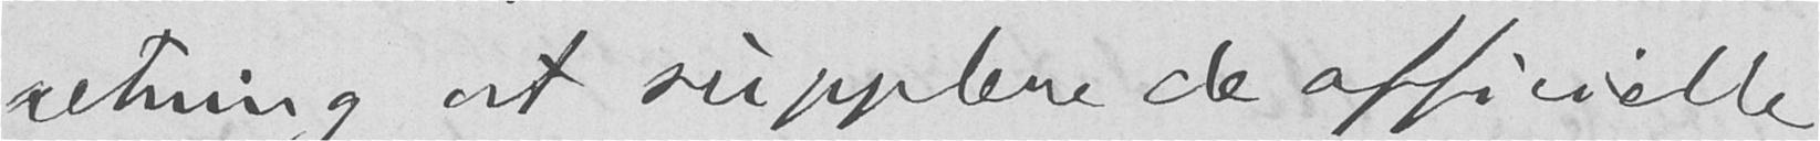retning at supplere de officielle
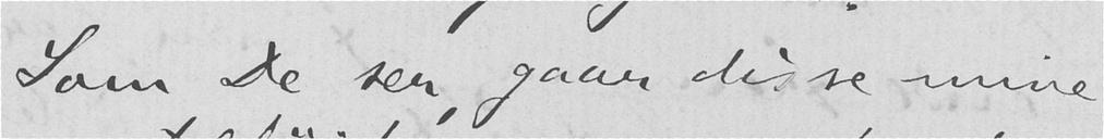Som De ser, gaar disse mine
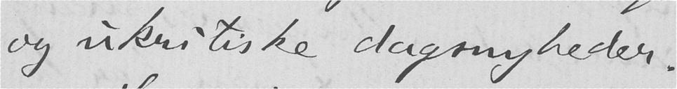og ukritiske dagsnyheder.
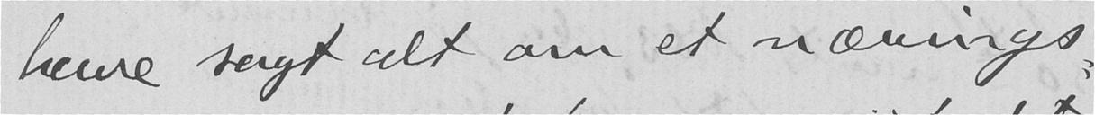have sagt alt om et nærings-
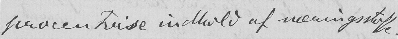procentvise indhold af næringsstoffe.
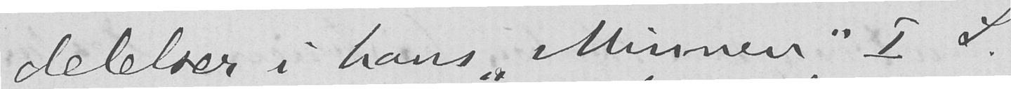delelser i hans "Minnen" I S.
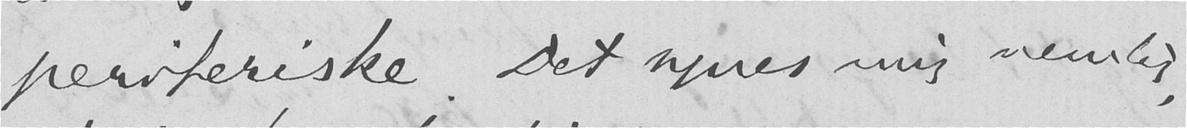periferiske. Det synes mig nemlig,
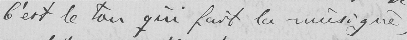C’est le ton qui fait la musique,
